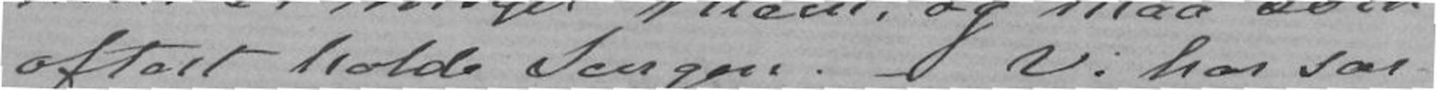aftalt holde Sengen. - Vi har ser-
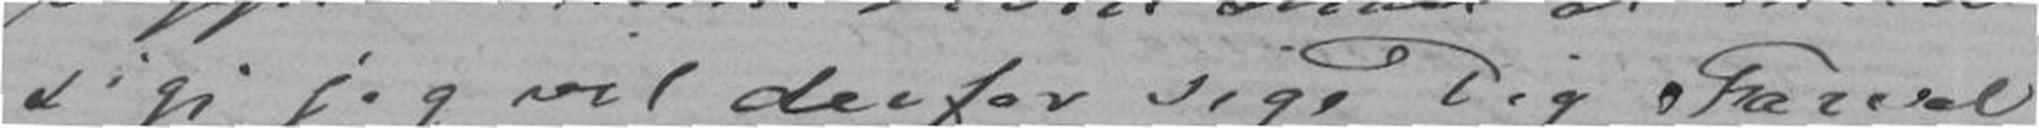sig; jeg vil derfor sige Dig Farvel
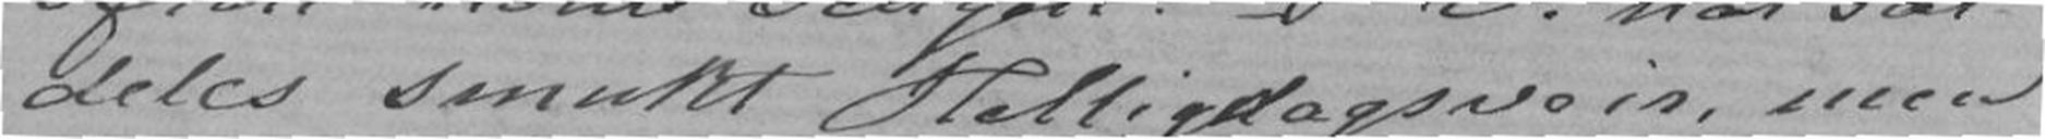deles smukt Helligdagsveir, men
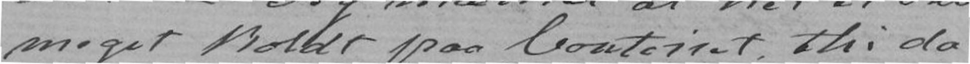meget koldt paa Contoiret, thi da
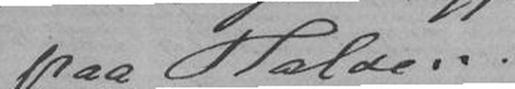paa Halsen.
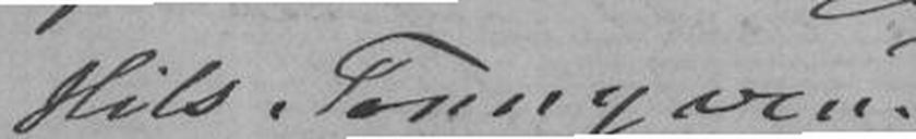Hils Tonny ven-
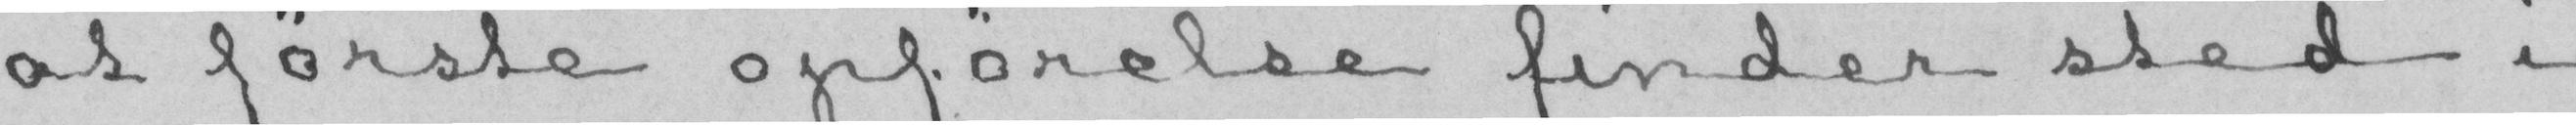at første opførelse finder sted i
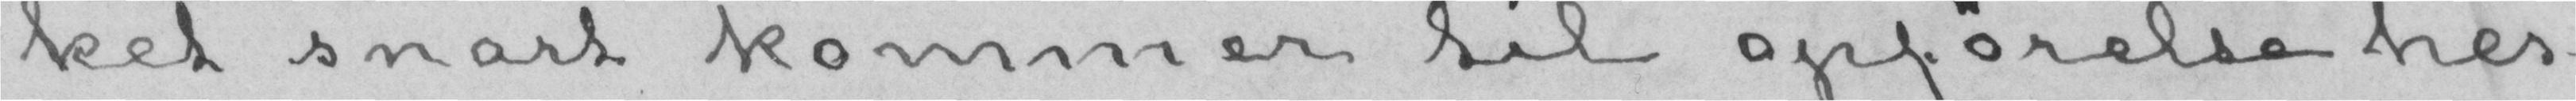ket snart kommer til opførelse her-
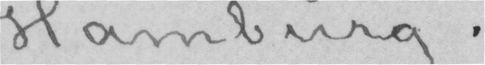Hamburg.
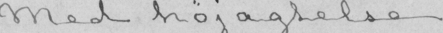Med højagtelse
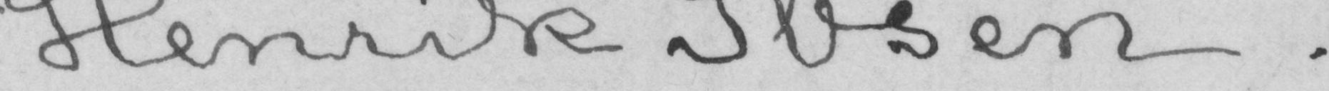Henrik Ibsen.
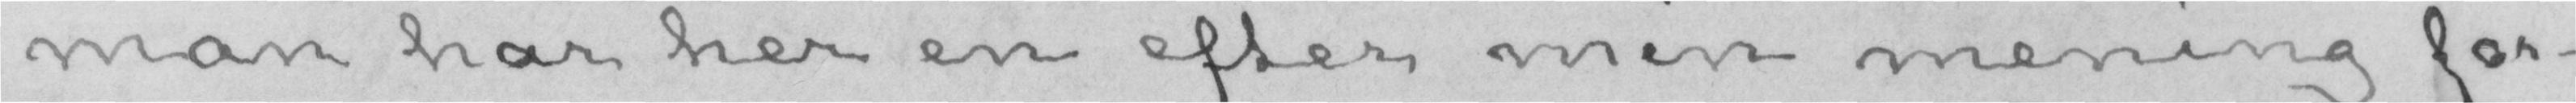man har her en efter min mening for-
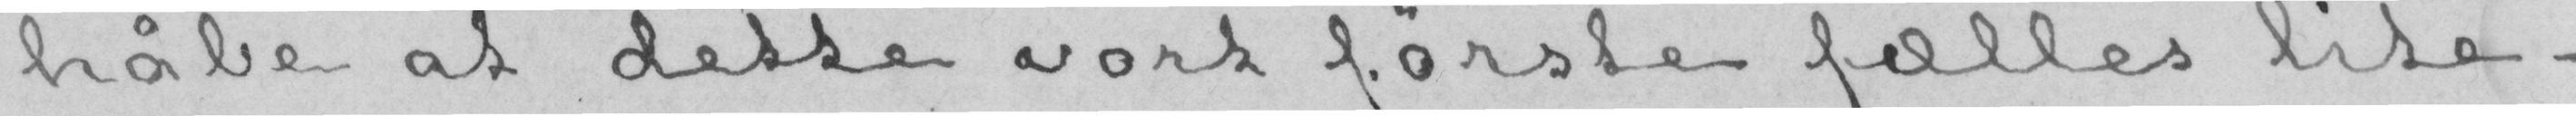håbe at dette vort første fælles lite-
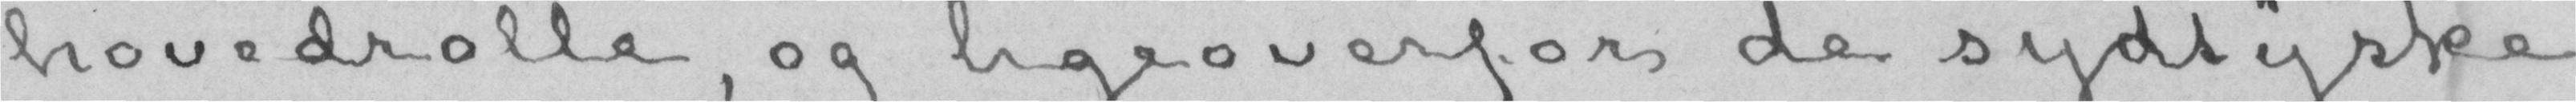hovedrolle, og ligeoverfor de sydtyske
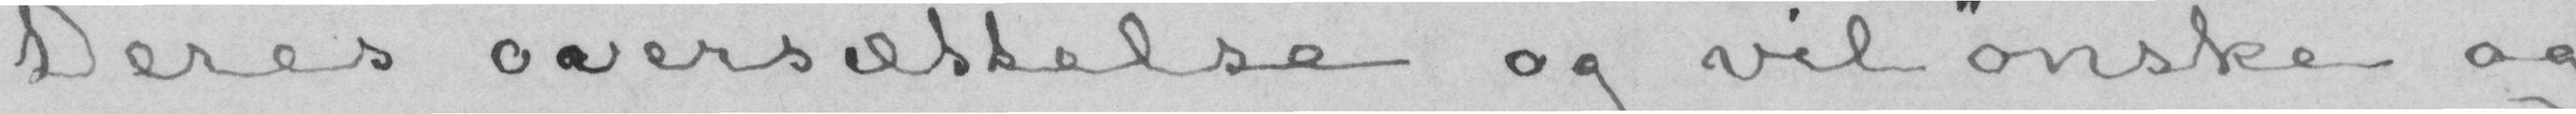Deres oversættelse og vil ønske og
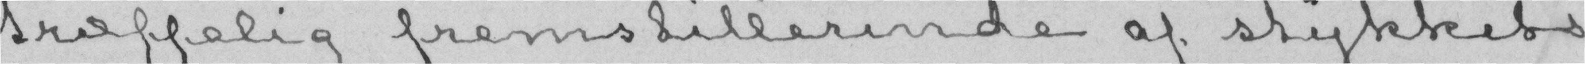træffelig fremstillerinde af stykkets
